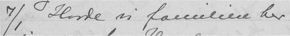7/1 Havde vi familien her
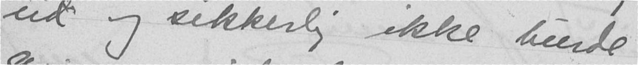ud og sikkerlig ikke burde
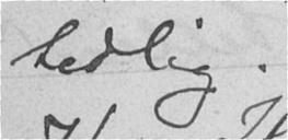tidlig.
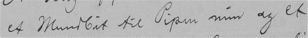et Mundbit til Pipen min og et
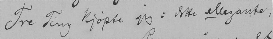Tre Ting kjøpte jeg: dette ellegante,
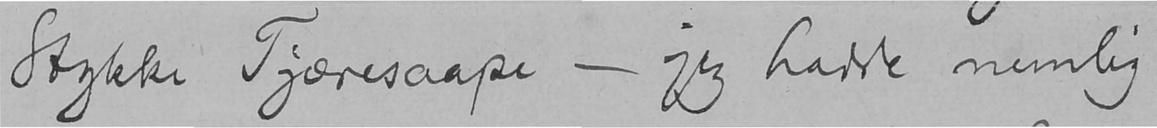Stykke Tjæresaape - jeg hadde nemlig
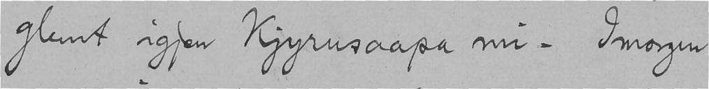glemt igjen Kjyrusaapa mi. Imorgen
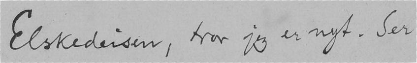Elskedeisen, tror jeg er nyt. Ser
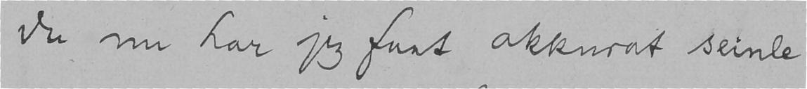du nu har jeg faat akkurat seinle
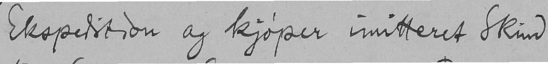Ekspedition og kjøper imitteret Skind
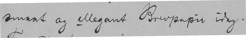smaat og ellegant Brevpapir idag.
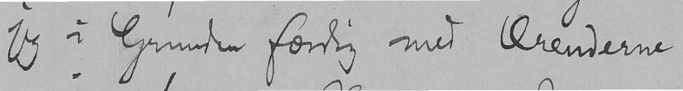jeg i Grunden færdig med Ærenderne
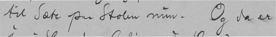til Sæte paa Stolen min. Og da er
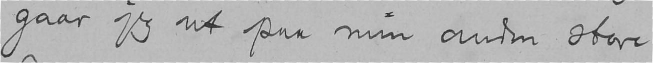gaar jeg ut paa min anden store
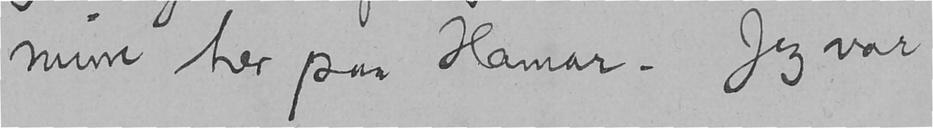mine her paa Hamar. Jeg var
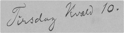Tirsdag Kvæld 10.
